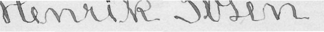Henrik Ibsen.
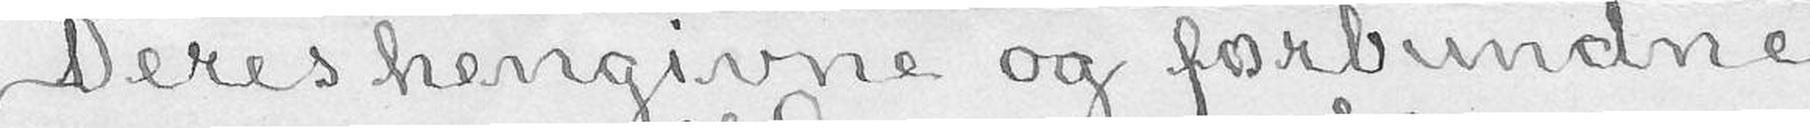Deres hengivne og forbundne
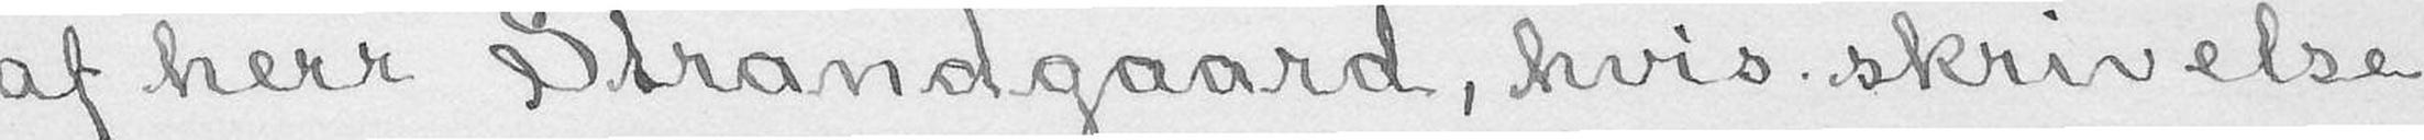af herr Strandgaard, hvis skrivelse
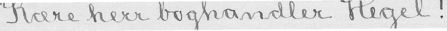Kære herr boghandler Hegel!
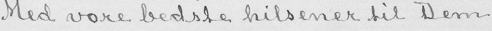Med vore bedste hilsener til Dem
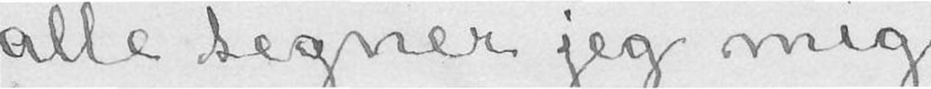alle tegner jeg mig
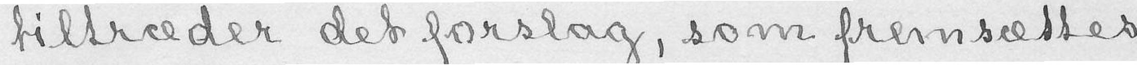tiltræder det forslag, som fremsættes
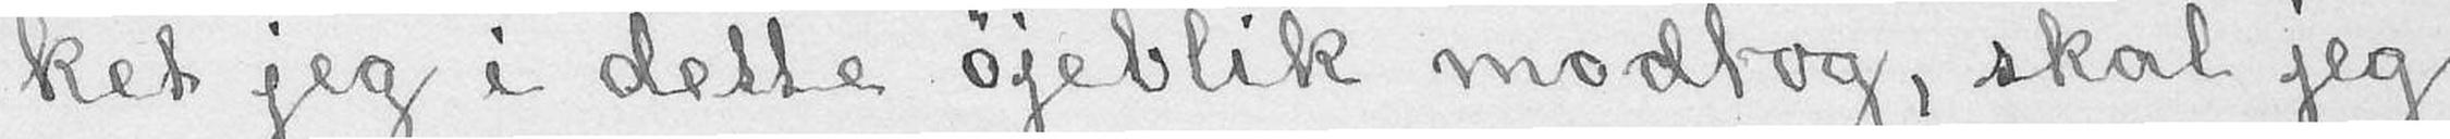ket jeg i dette øjeblik modtog, skal jeg
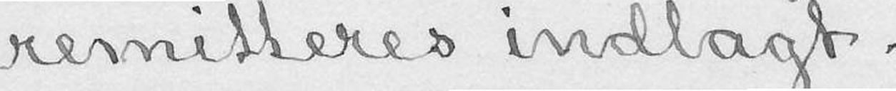remitteres indlagt.
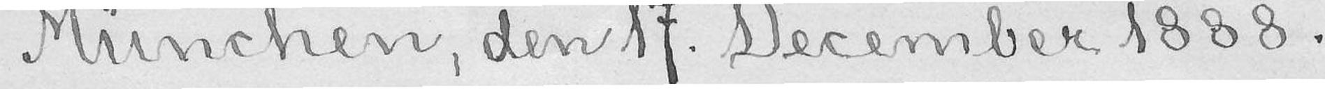München, den 17. December 1888.
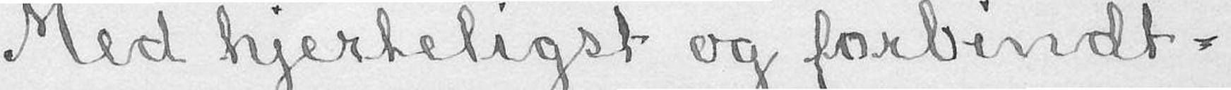Med hjerteligst og forbindt-
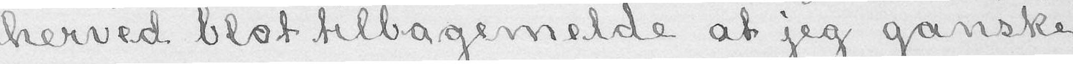herved blot tilbagemelde at jeg ganske
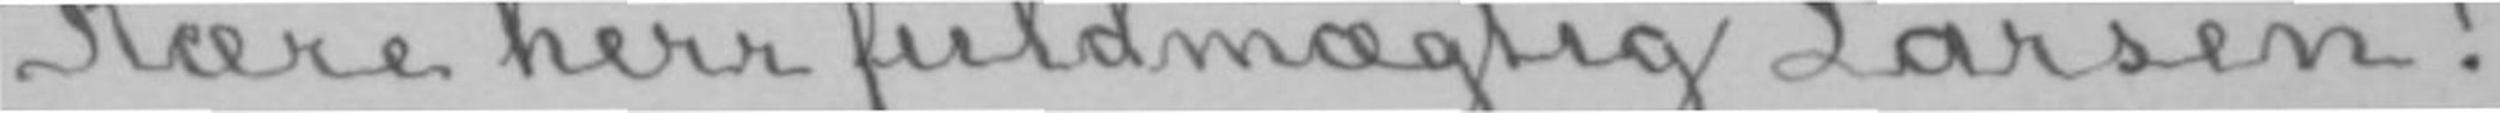Kære herr fuldmægtig Larsen!
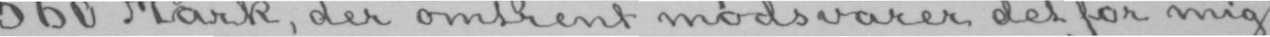560 Mark, der omtrent modsvarer det for mig
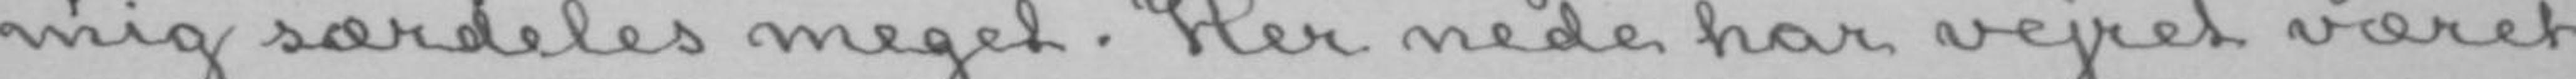mig særdeles meget. Her nede har vejret været
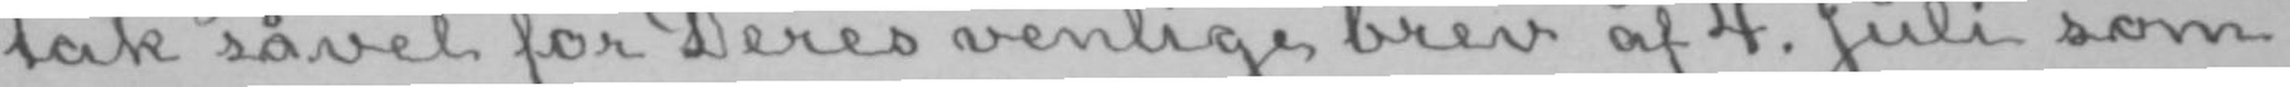tak såvel for Deres venlige brev af 4. Juli som
